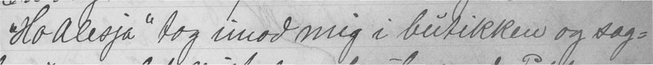"Ho Alesja" tog imod mig i butikken og sag-
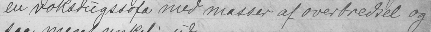en voksdugssofa med masser af overbredsel og
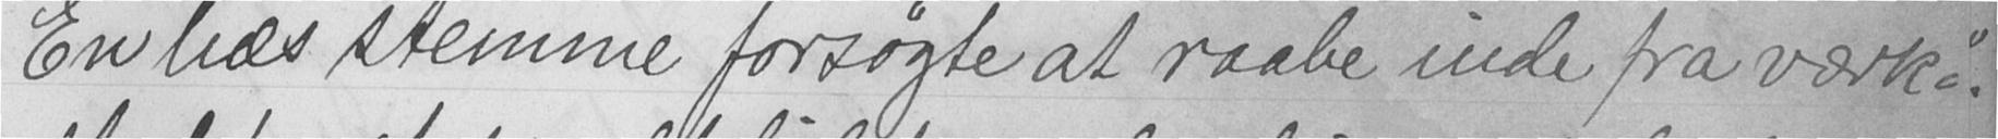En hæs stemme forsøgte at raabe inde fra værk-
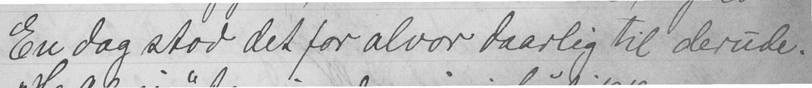En dag stod det for alvor daarlig til derude.
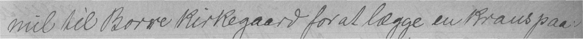mil til Borre kirkegaard for at lægge en krans paa
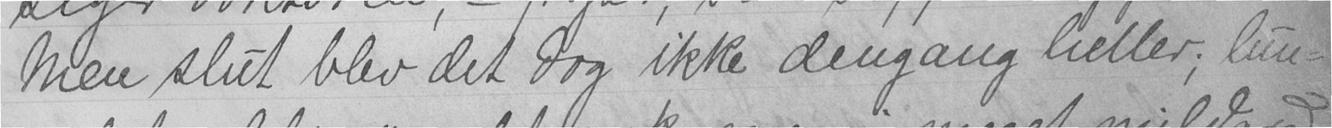Men slut blev det dog ikke dengang heller; lun-
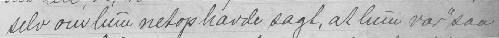selv om hun netop havde sagt, at hun var "saa
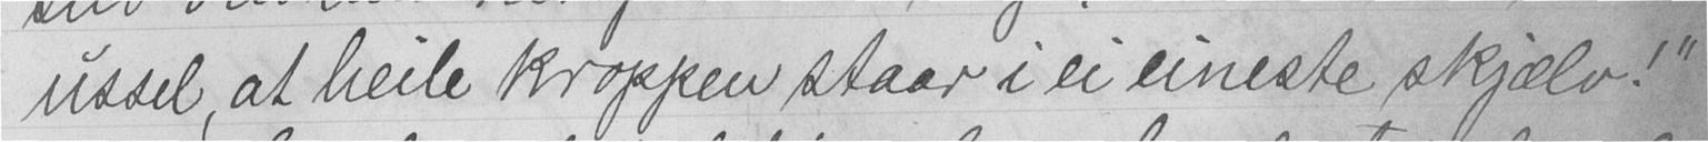ussel, at heile kroppen staar i ei eineste skjælv!"
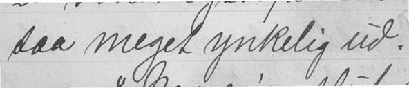saa meget ynkelig ud.
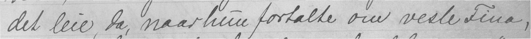det leie, da, naarhun fortalte om vesle Tina,
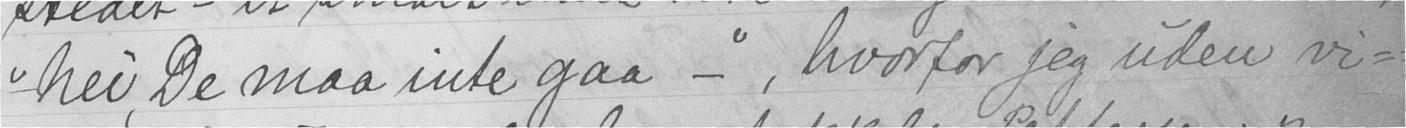"Nei, De maa inte gaa -" , hvorfor jeg uden vi-
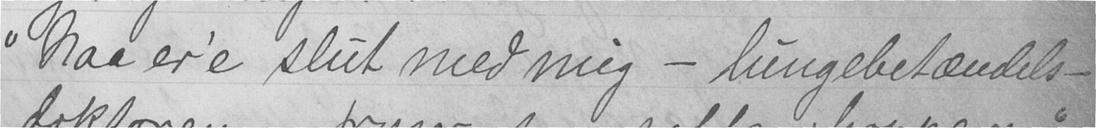"Naa er 'e slut med mig - lungebetændels -
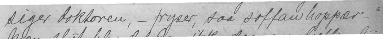siger doktoren, - fryser, saa soffan hoppær - "
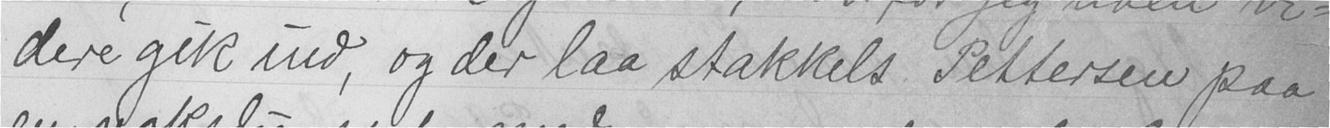dere gik ind, og der laa stakkels Pettersen paa
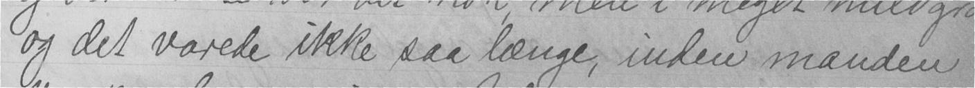og det varede ikke saa længe, inden manden
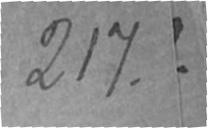217.
